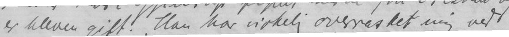er bleven gift. Han har virkelig overrasket mig ved
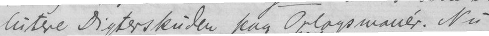lutere Digterskuden paa Orlogsmanér. Nu
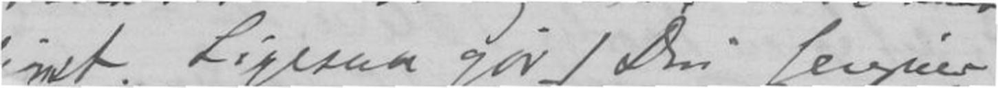Ligesaa gør Din hengivne
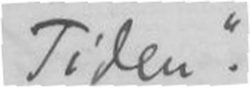Tiden".
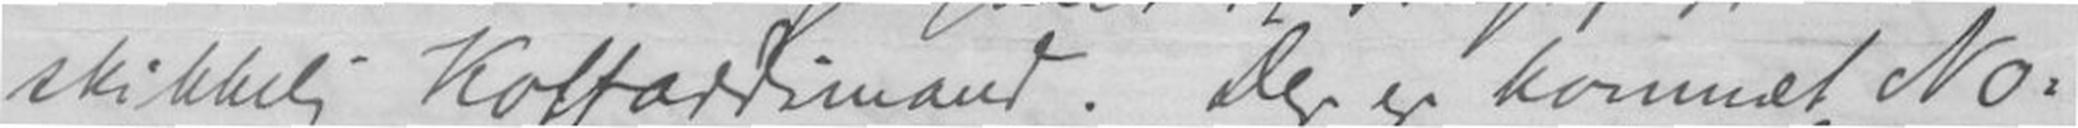skikkelig Koffærdimand. Der er kommet No-
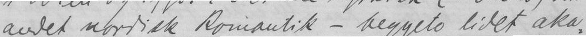andet nordisk Romantik - beggeto lidet aka-
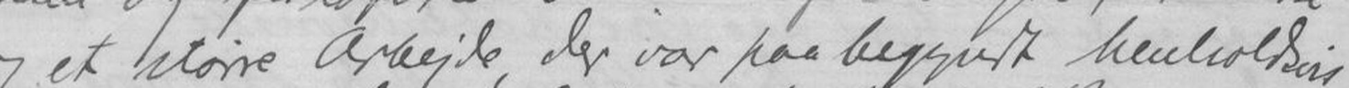og et større Arbejde, der var paabegyndt henholdsvis
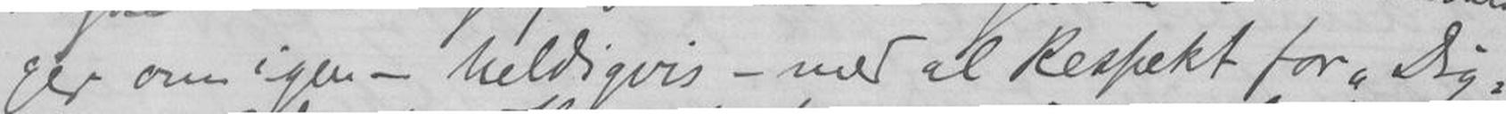ger? om igen - heldigvis - med al Respæktfor "Dig-
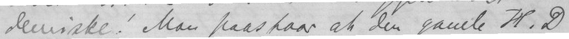demiske! Man paastaar at den gamle H.D.
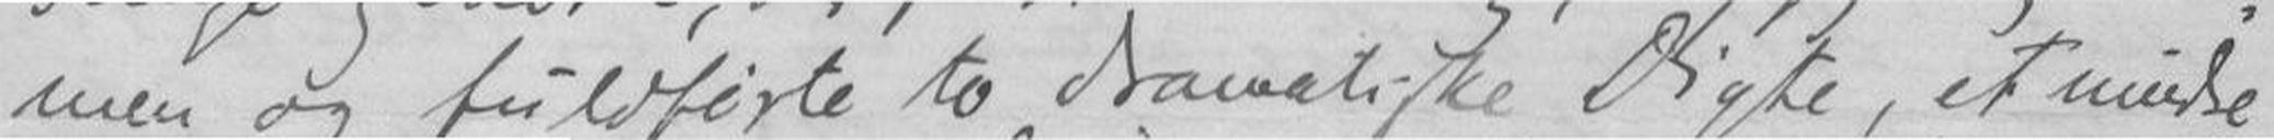men og fuldførte to Dramatiske Digte, et mindre
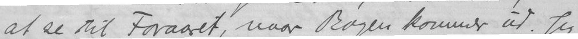at se til Foraaret, naar Bogen kommer ud. Jeg
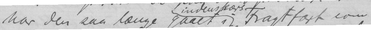har den saa længe gaaet i Fragtfart som
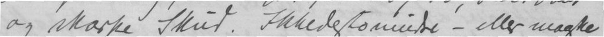og skarpe Skud. Ikkedestomindre - eller magte
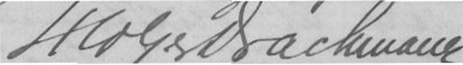Holger Drackmann
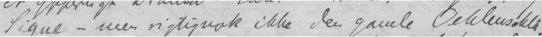Signe - men rigtignok ikke den gamle Ochlenscklä-
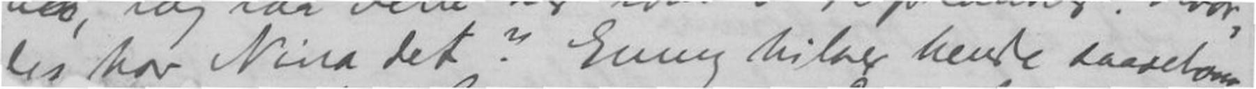har Nina det? Emmy hilser hende taadelom
